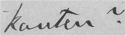kanten?
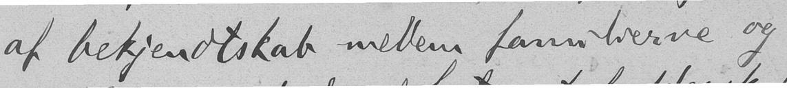af bekjendtskab mellem familierne og
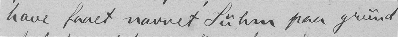have faaet navnet Suhm paa grund
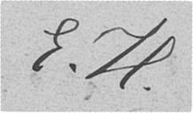E. H.
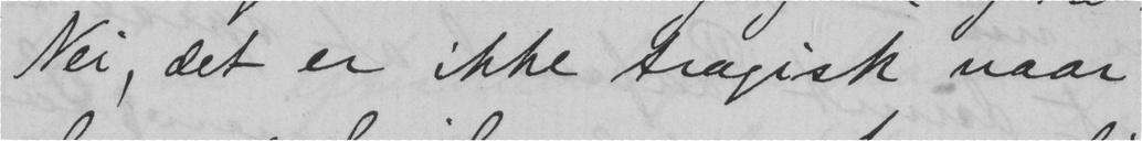Nei, det er ikke tragisk naar
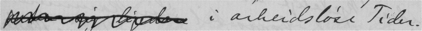i arbeidsløse Tider.
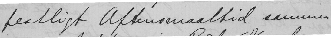festligt Aftensmaaltid sammen
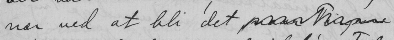nær ved at bli det
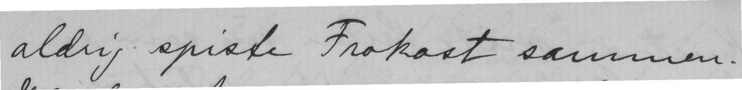aldrig spiste Frokast sammen.
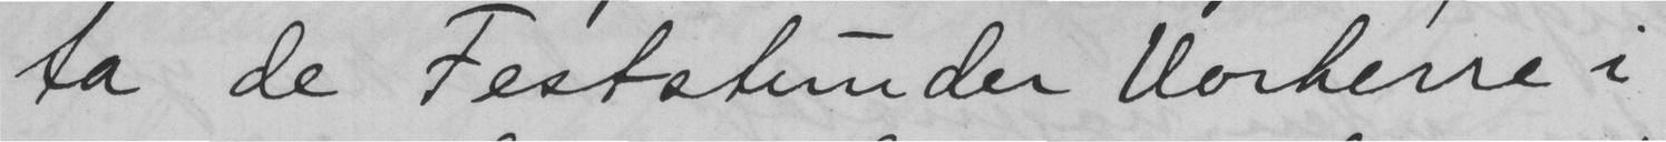ta de Feststunder Vorherre i
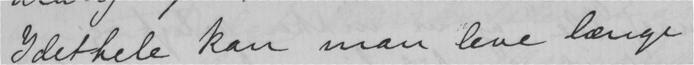I det hele kan man leve længe
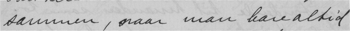sammen, naar man bare altid.
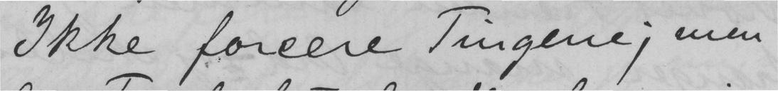Ikke forcere Tingene; men
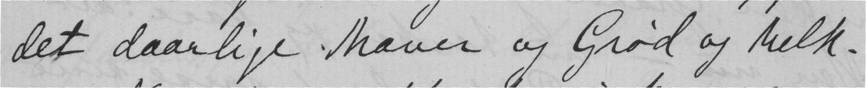det daarlige Maver og Grød og Melk.
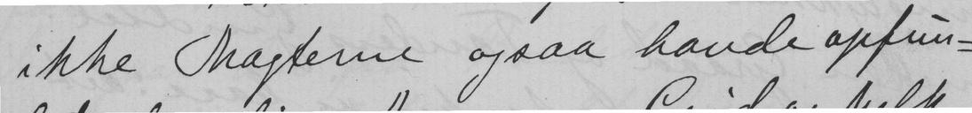ikke Magterne ogsaa havde opfun-
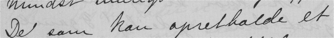De som kan opretholde et
